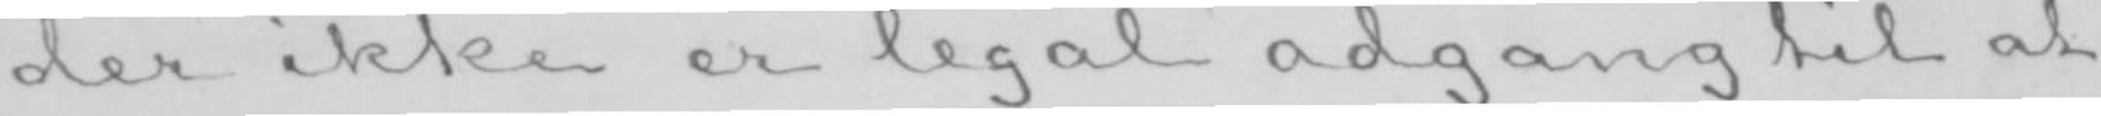der ikke er legal adgang til at
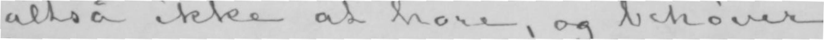altså ikke at høre, og behøver
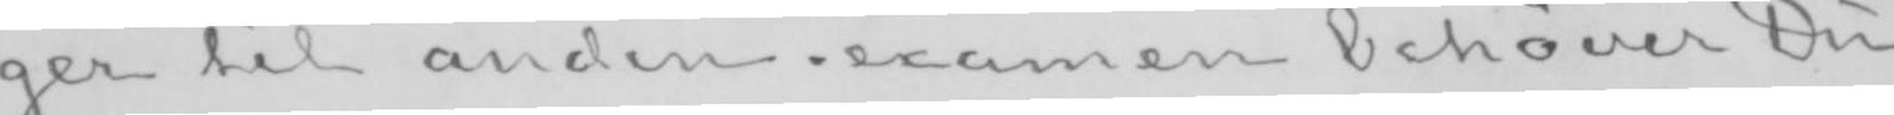ger til anden-examen behøver Du
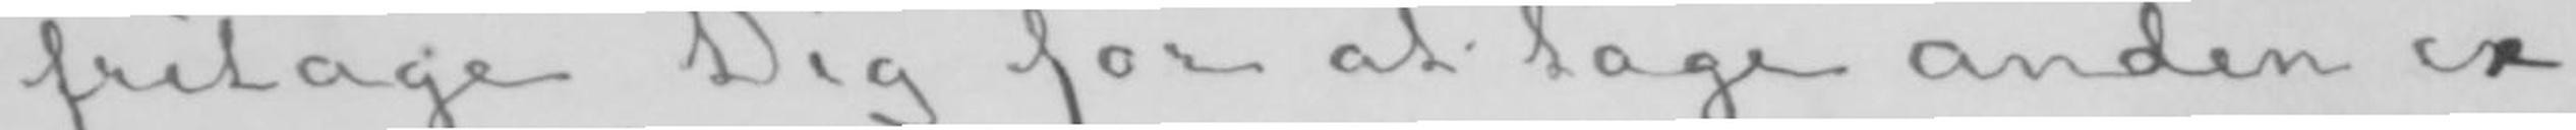fritage Dig for at tage anden ex-
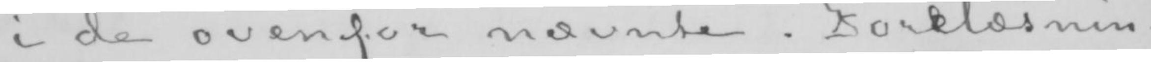i de ovenfor nævnte. Forlelæsnin-
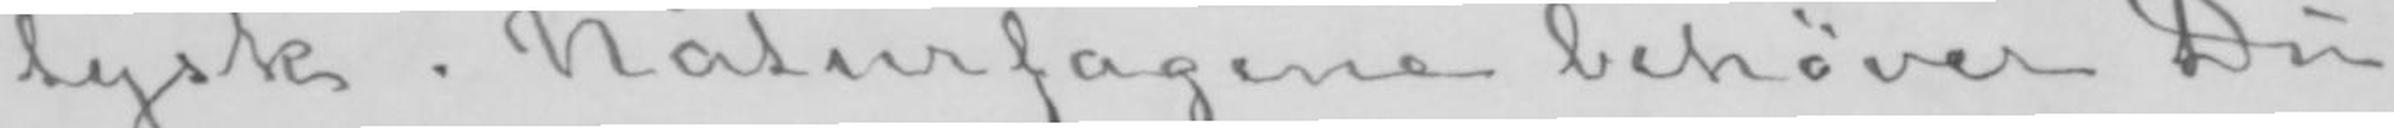tysk. Naturfagene behøver Du
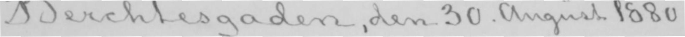Berchtesgaden, den 30. August 1880.
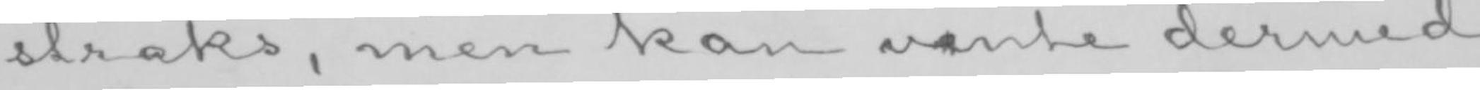straks, men kan vente dermed
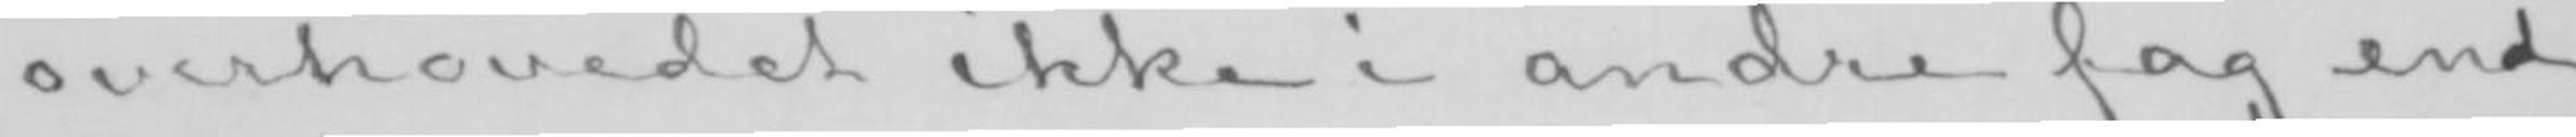overhovedet ikke i andre fag end
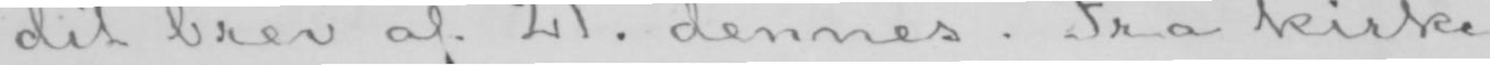dit brev af 21. dennes. Fra kirke-
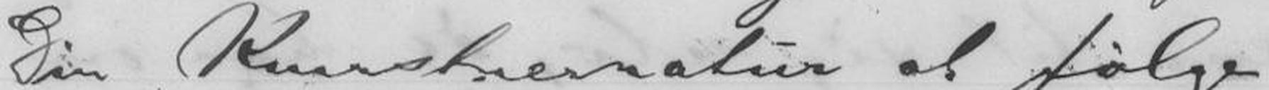Din Kunstnernatur at følge
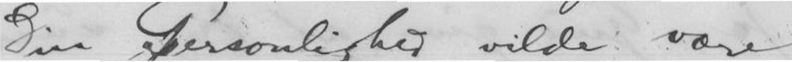Din Personlighed vilde være
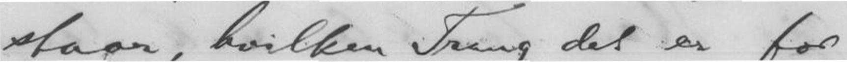staar, hvilken Trang det er for
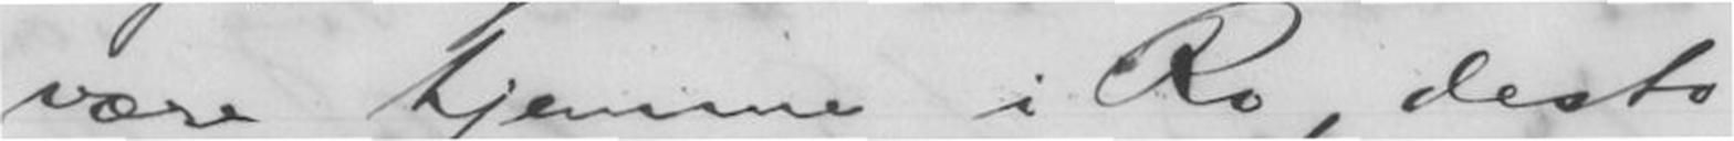være hjemme i Ro, desto
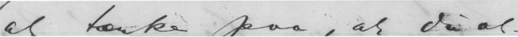at tænke paa, at Du at-
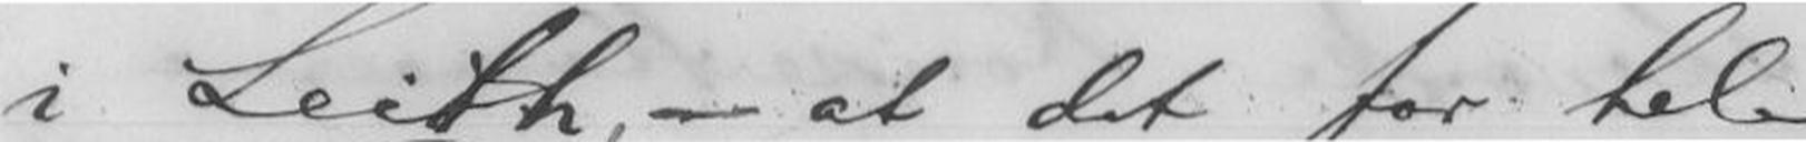i Leith, - at det for hele
